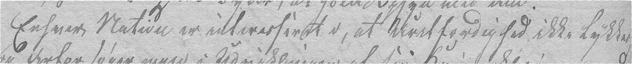Enhver Nation er interesseret i, at Uretfærdighed ikke lykkes
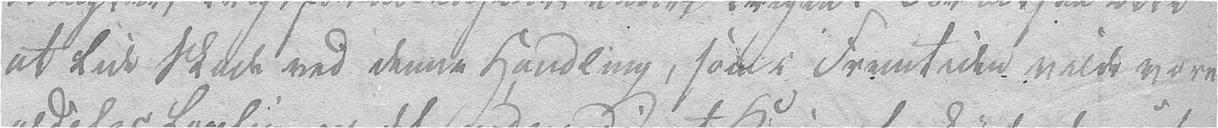at lide Skade ved denne Handling, som i Fremtiden vilde være
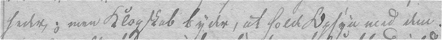heder; men Klogskab byder, at holde Opsyn med dem.
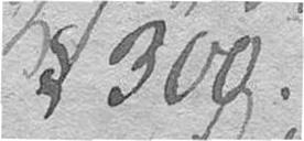§ 300.
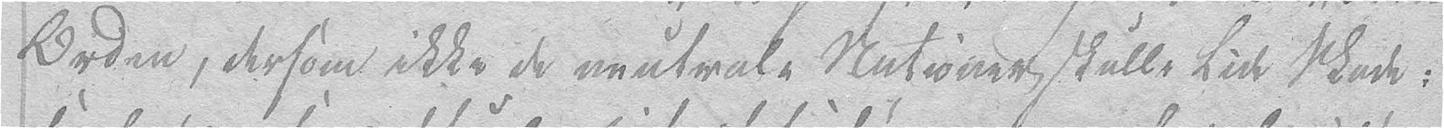Orden, dersom ikke de neutrale Nationer skulle lide Skade:
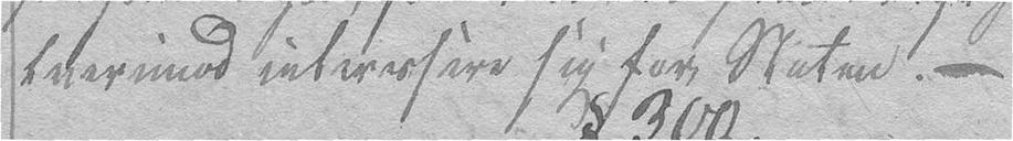tverimod interessere sig for Staten. –
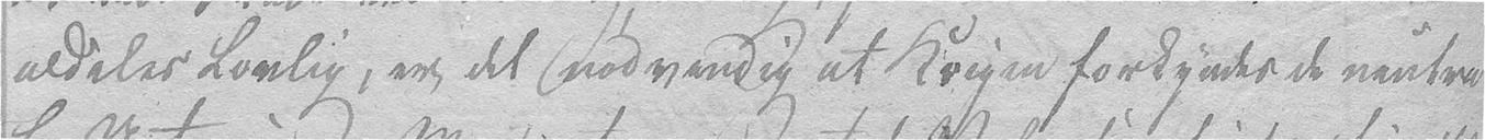aldeles Lovlig, er det nødvendig at Krigen forkyndes de neutra-
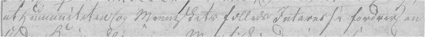at Humaniteten og Menneskets fælleds Interesse fordrer en
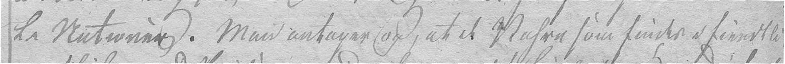le Nationer. Man antager og, at de Vahre som findes i fiendtli-
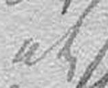at
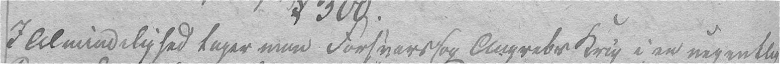I Almindelighed tager man Forsvars og Angrebs Krig i en uegentlig
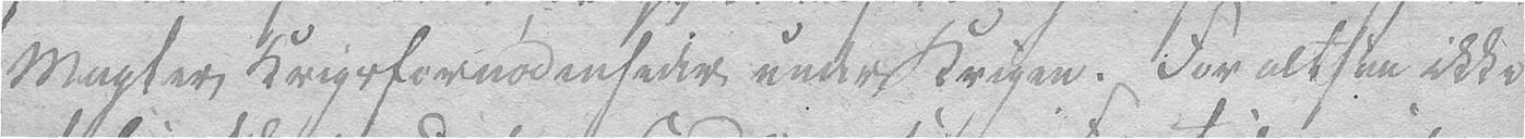Magter Krigsfornødenheder under Krigen. For altsaa ikke
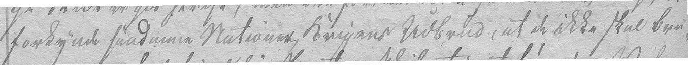forkynde saadanne Nationer Krigens Udbrud, at de ikke skal bru-
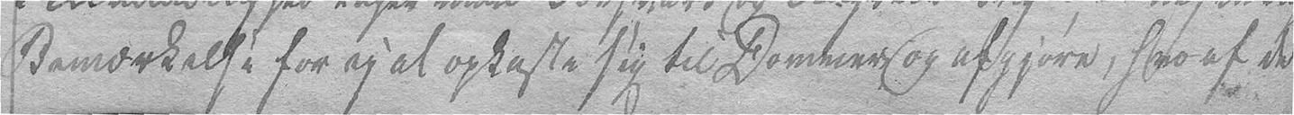Bemærkelse for ey at opkaste sig til Dommer og afgjøre, hvo af de
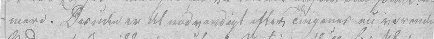mere. Desuden er det nødvendigt efter Tingenes nu værende
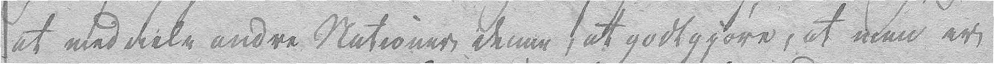at meddele andre Nationer denne, at godtgjøre, at man er
